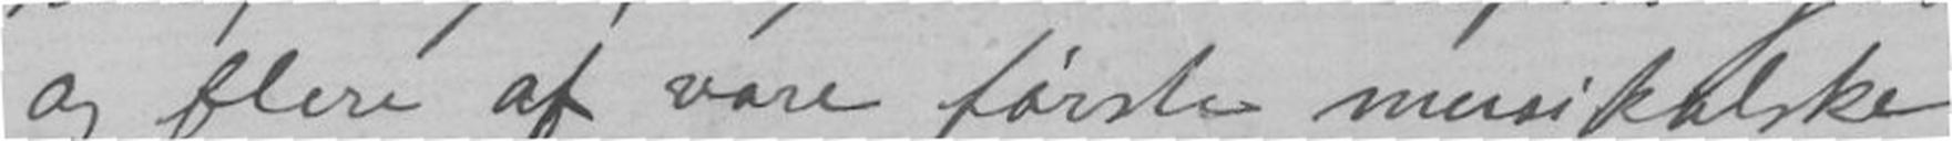og flere af vore første musikalske
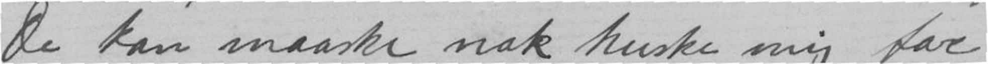De kan maaske nok huske mig for
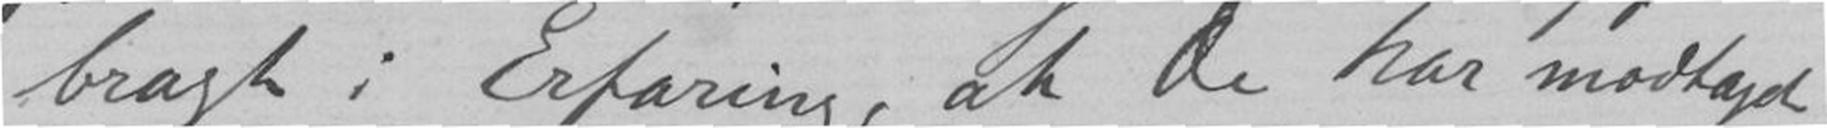bragt i Erfaring, at De har modtaget
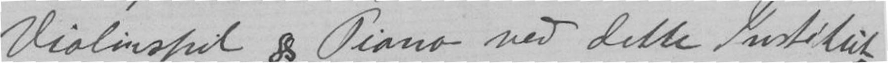Violinspil & Piano ved dette Institut.
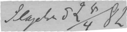Slagelse d 28/4 82
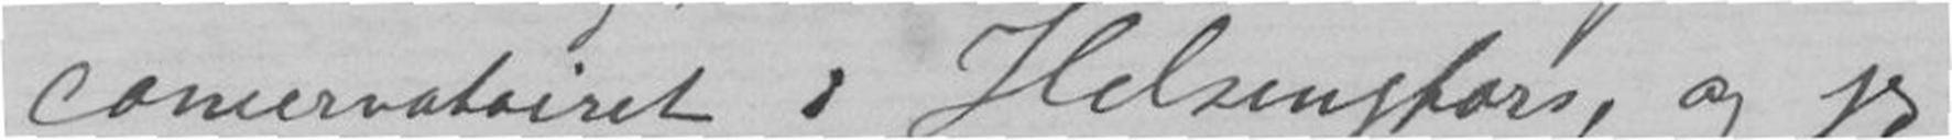=concervatoriet i Helsingfors, og jeg
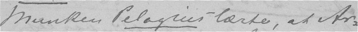Munken Pelagius lærte, at Ar-
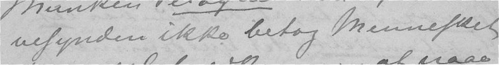vesynden ikke betog Mennesket
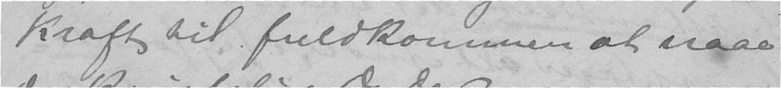Kraft til fuldkommen at naae
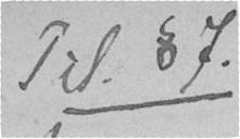Til § 7.
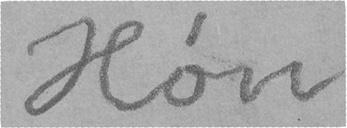Høn
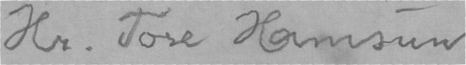Hr. Tore Hamsun
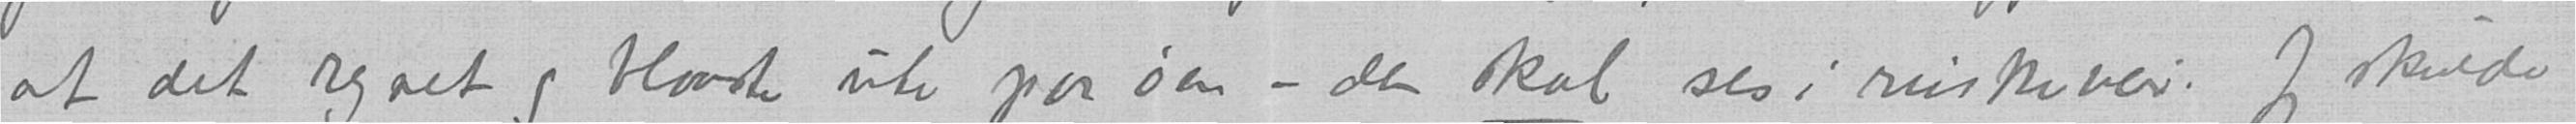at det regnet og blaaste ute paa øen - den skal ses i ruskeveir. Jeg skulde
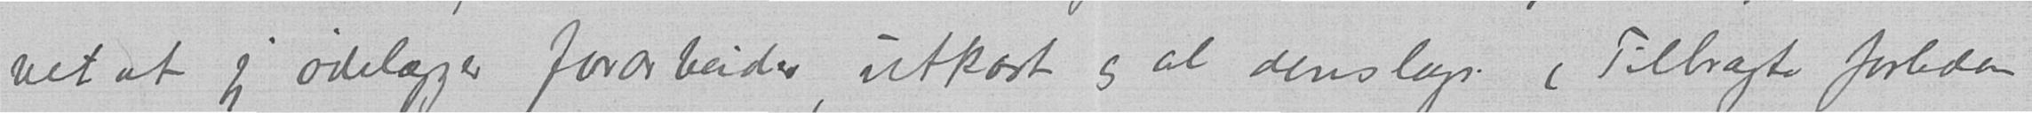vet at jeg ødelægger forarbeider, utkast og al denslags. (Tilbragte forleden
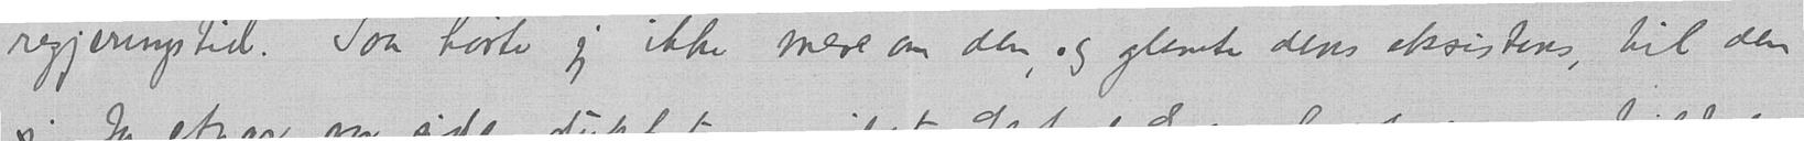regjeringstid. Saa hørte jeg ikke mere om den, og glemte dens eksistens, til den
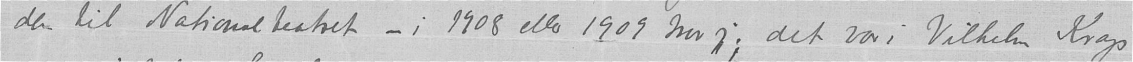den til Nationalteatret – i 1908 eller 1909 tror jeg, det var i Vilhelm Krags
